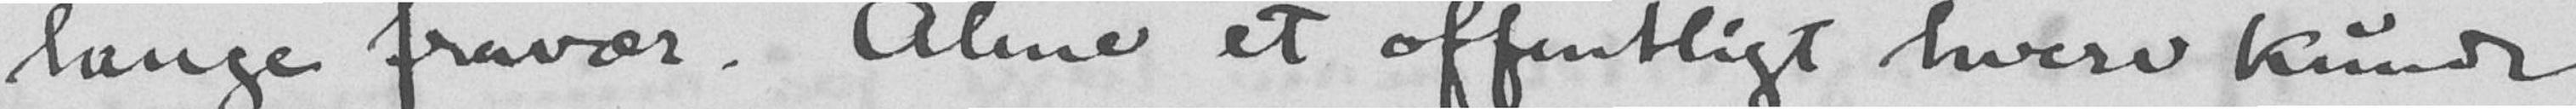lange fravær. Alene et offentligt hverv kunde
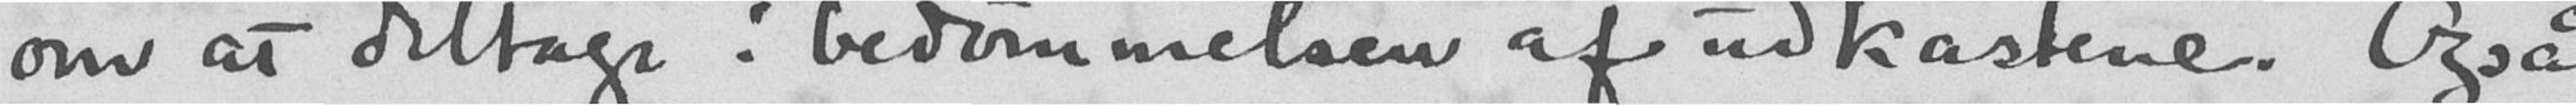om at deltage i bedømmelsen af udkastene. Også
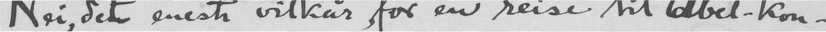Nei, det eneste vilkår for en reise til Abel-kon
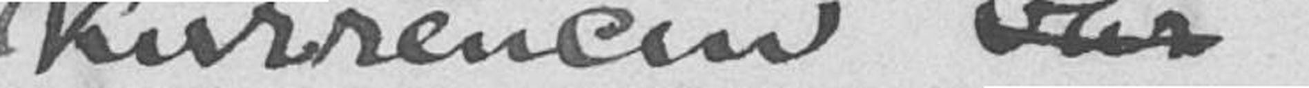kurrencen var
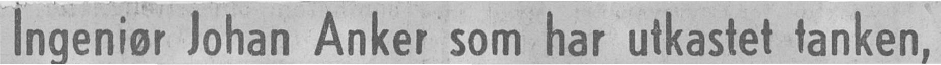Ingeniør Johan Anker som har utkastet tanken,
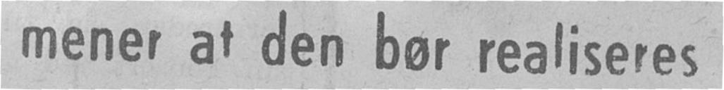mener at den bør realiseres
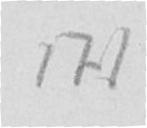171
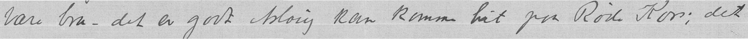bare bra – det er godt Aslaug kan komme hit paa Røde Kors; det
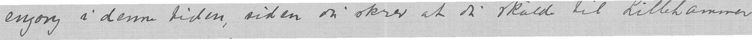engang i denne tiden, siden du skrev at du skulde til Lillehammer
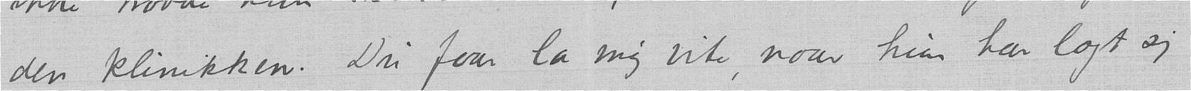den klinikken. Du faar la mig vite, naar hun har lagt sig
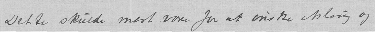Dette skulde mest være for at ønske Aslaug og
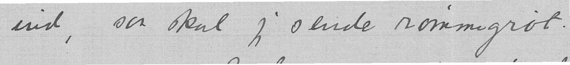ind saa skal jeg sende rømmegrøt.
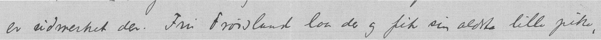er udmerket der. Fru Frøisland laa der og fik sin ældste lille pike,
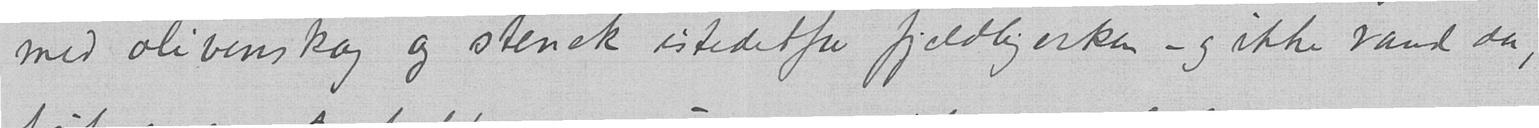med olivenskog og stenek istedetfor fjeldbjerken – og ikke vand da,
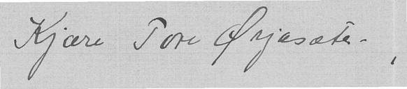Kjære Tore Ørjasæter,
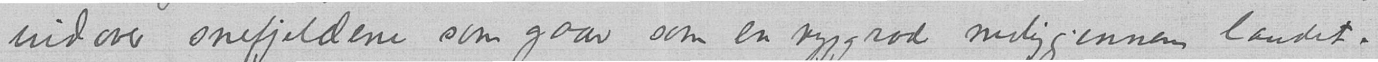indover snefjeldene som gaar som en ryggrad nediggennem landet.
